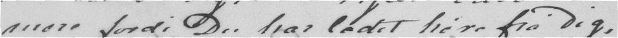mere fordi Du har ladet høre fra Dig,
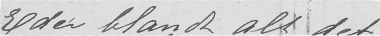Eder blandt alt det
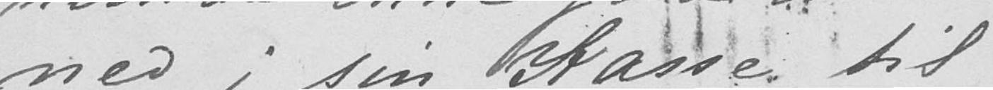ned i sin Kasse til
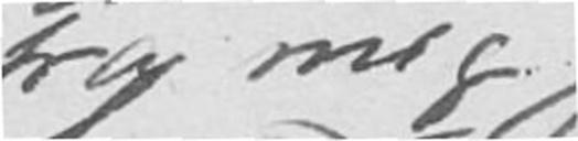fra mig
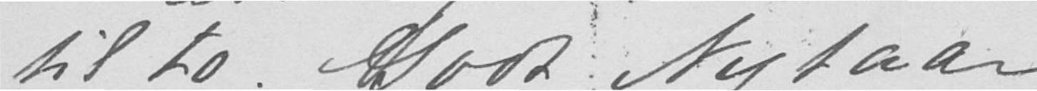til to. Godt Nytaar
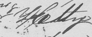Kitty
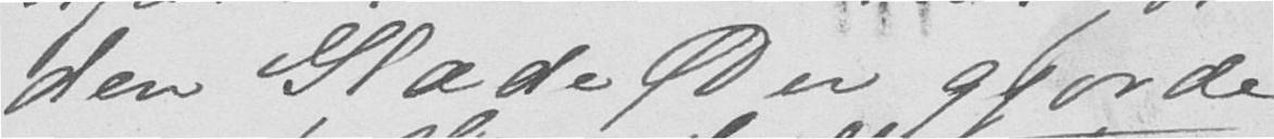den Glæde Du gjorde
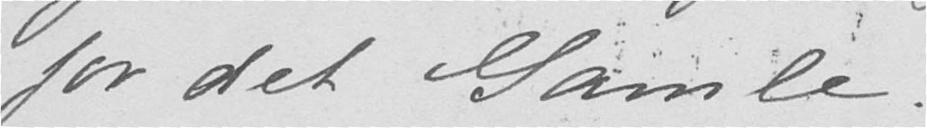for det Gamle.
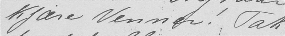Kjære Venner! Tak
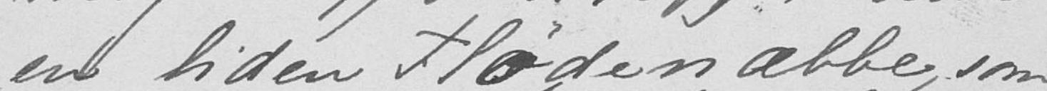en liden Flødenæbbe, som
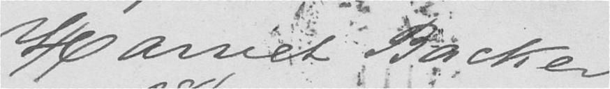Harriet Backer
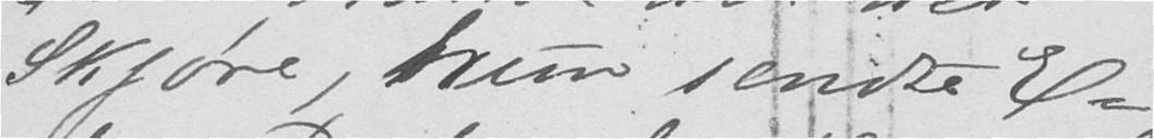Skjøre, hun sendte E-
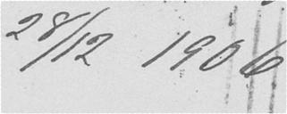28/12 1906.
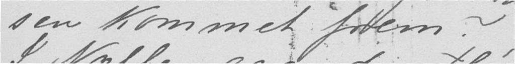sen kommet frem?
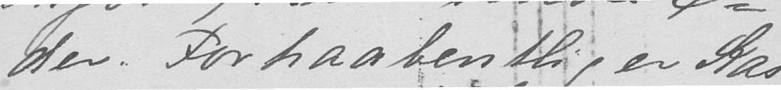der. Forhaabentlig er Kas-
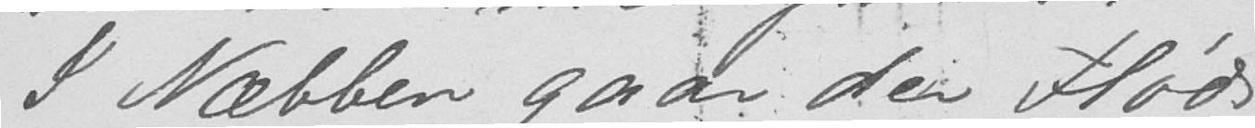I Næbben gaar der Fløde
